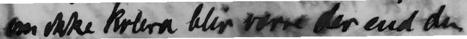- om ikke kolera blir værre der end den
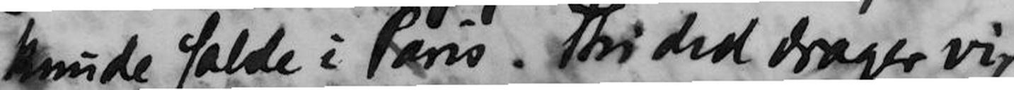kunde falde i Paris. Thi did drager vi,
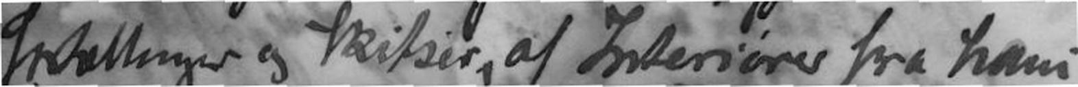fortællinger og Skitser, af Interiører fra hans
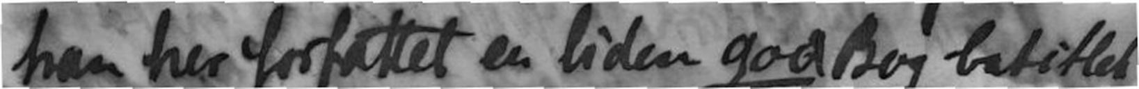han her forfattet en liden god Bog betitlet
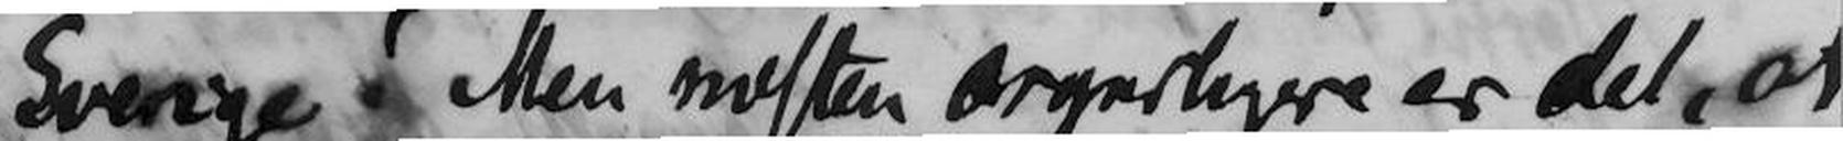Sverige! Men næsten ærgerligere er det, os
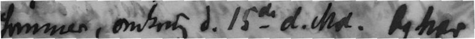Sommer, omkring d. 15de d. Md. Og har
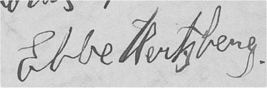Ebbe Hertzberg.
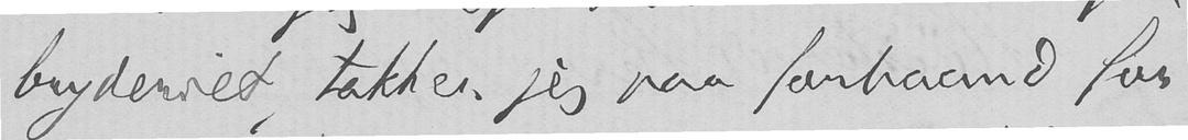bryderiet, takker jeg paa forhaand for
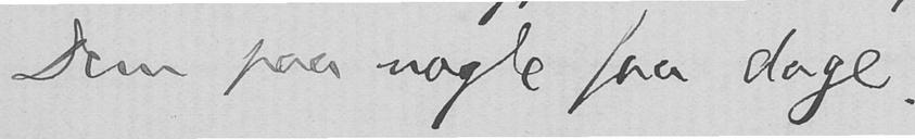Dem paa nogle faa dage.
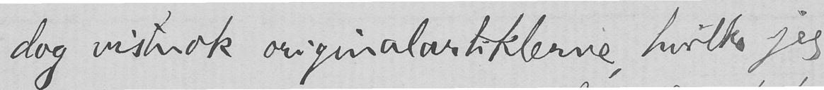dog vistnok originalartiklerne, hvilke jeg
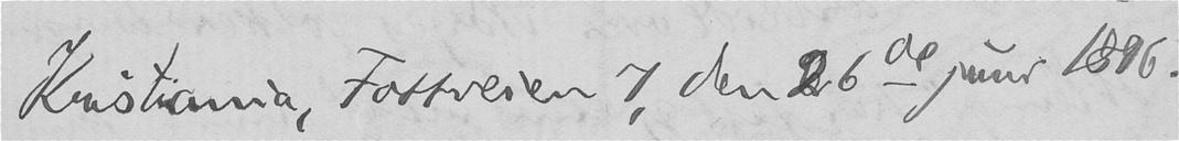Kristiania, Fossveien 7, den 26de juni 1896.
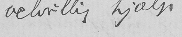velvillig hjælp.
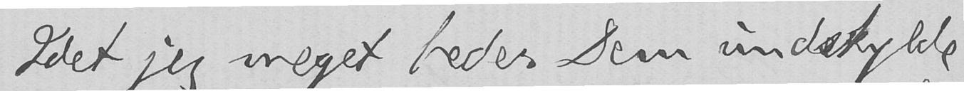Idet jeg meget beder Dem undskylde
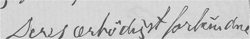Deres ærbødigst forbundne
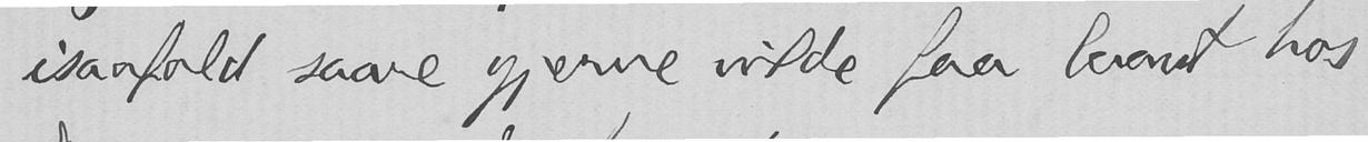isaafald saare gjerne vilde faa laant hos
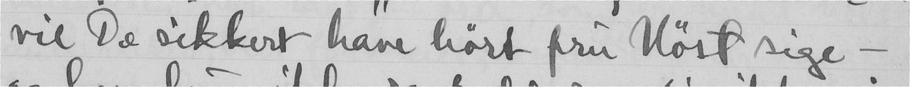vil De sikkert have hørt fru Høst sige
